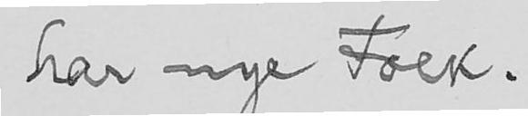har nye Folk.
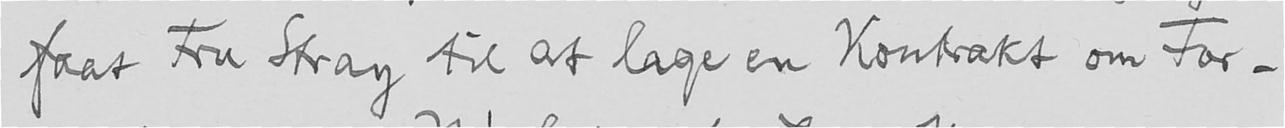faat Fru Stray til at lage en Kontrakt om For-
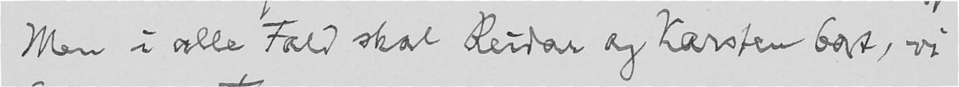Men i alle Fald skal Reidar og Karsten bort, vi
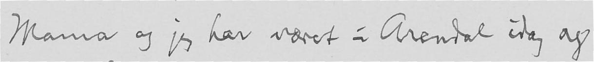Mama og jeg har været i Arendal idag og
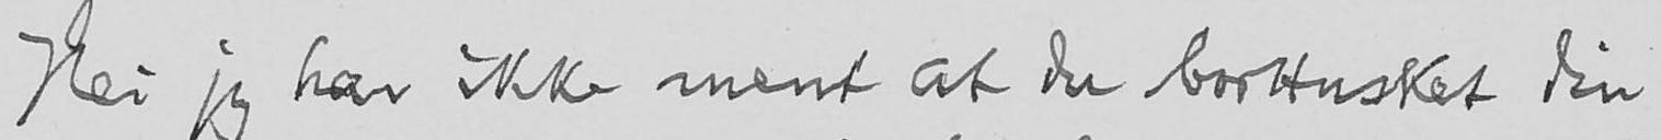Nei jeg har ikke ment at du borttusket din
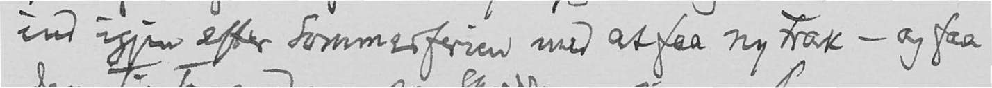ind igjen efter Sommerferien med at faa ny Frak - og faa
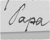Papa
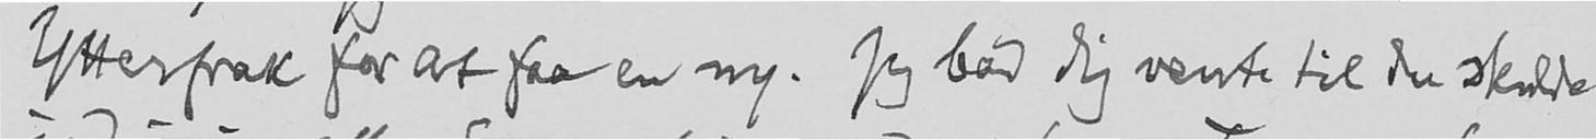Ytterfrak for at faa en ny. Jeg bad dig vente til du skulde
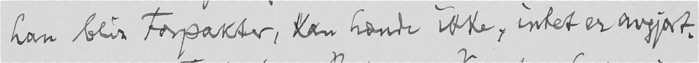han blir Forpakter, kan hænde ikke, intet er avgjort.
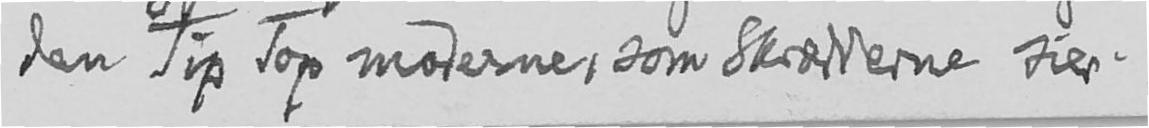den Tip Top moderne, som Skrædderne sier.
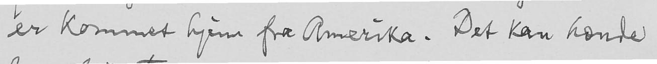er kommet hjem fra Amerika. Det kan hænde
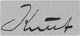Knut
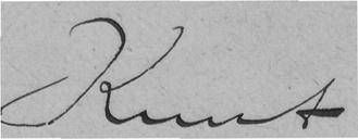Knut
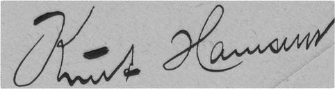Knut Hamsun
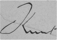Knut
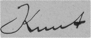Knut
